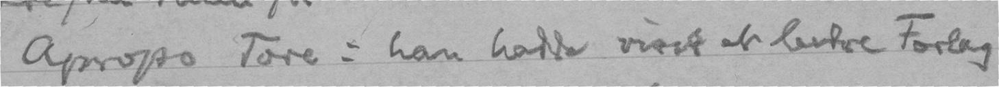Apropo Tore: han hadde visst et bedre Forlag
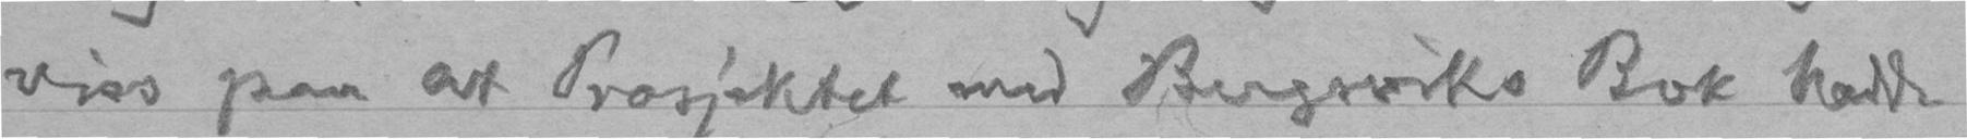viss paa at Prosjektet med Bergsviks Bok hadde
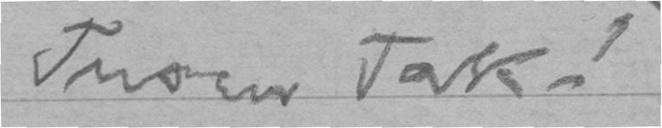Tusen Tak!
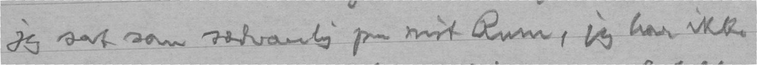jeg sat som sædvanlig paa mit Rum, jeg har ikke
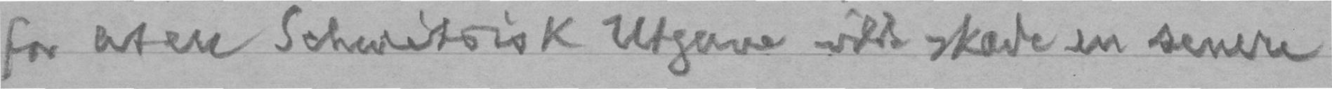for at en Schweitsisk Utgave vilde skade en senere
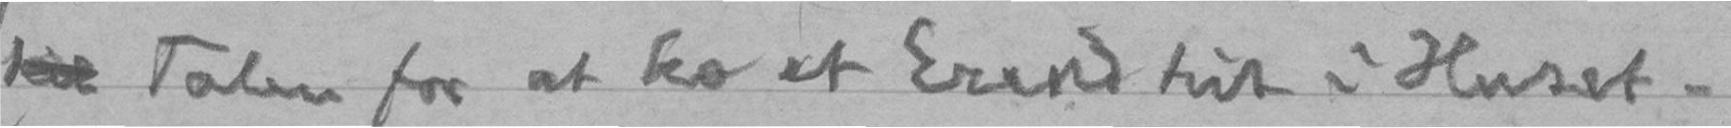til Talen for at ha et Erend hit i Huset.
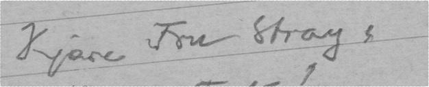Kjære Fru Stray,
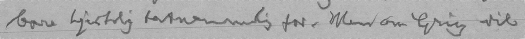bare hjertelig taknemmelig for. Men om Grieg vil
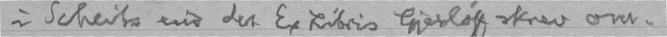i Scheits end det Ex Libris Gierløff skrev om.
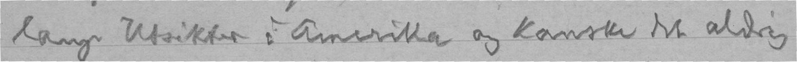lange Utsikter i Amerika og kanske det aldrig
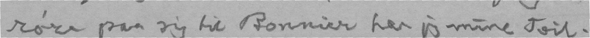røre paa sig til Bonnier har jeg mine Tvil.
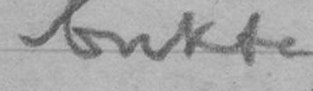brukte
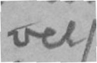vel
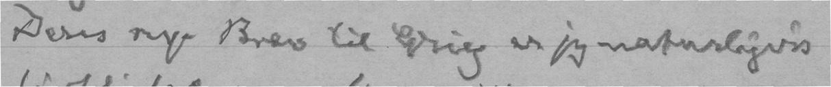Deres nye Brev til Grieg er jeg naturligvis
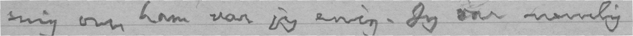mig om ham var jeg enig. Jeg var nemlig
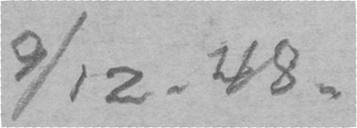9/12. 48.
